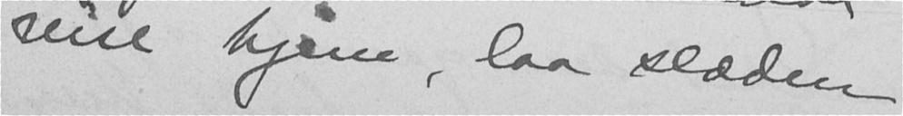seile hjem, laa slæden
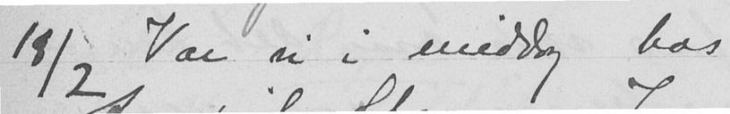18/2 Var vi i middag hos
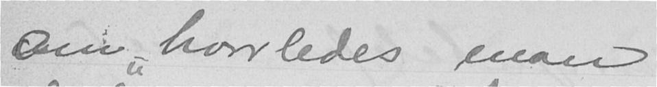om "hvorledes man
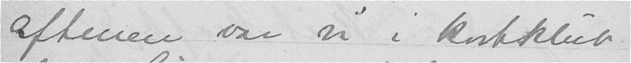aftenen var vi i kortklub
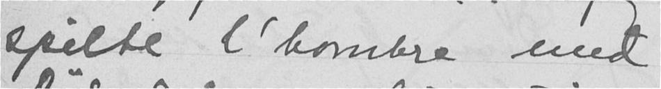spilte l'hombre med
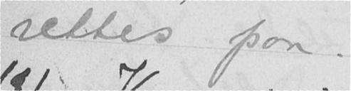rettes paa.
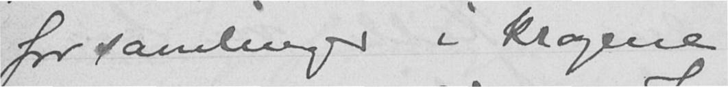forsamlinger i krogene
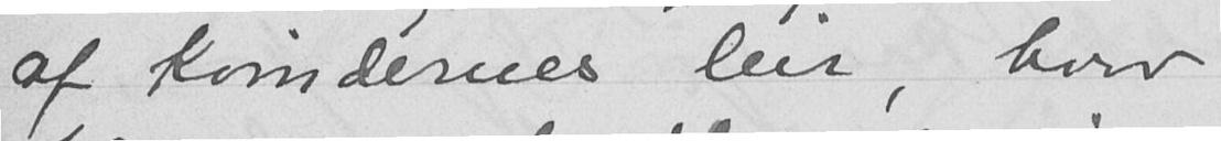af kvindernes leir, hvor
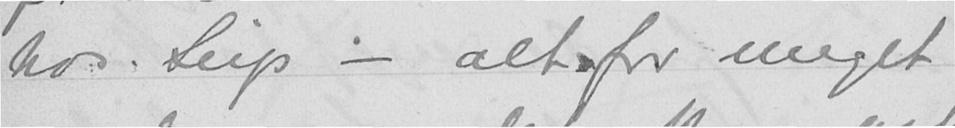hos Seip - altfor meget
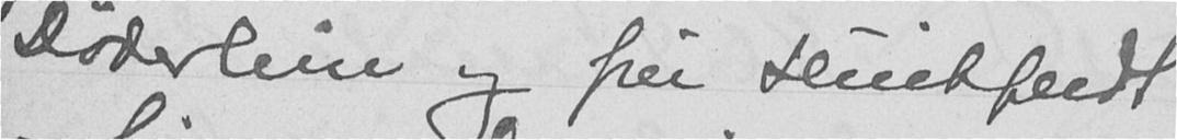Døderlein og fru Huitfeldt
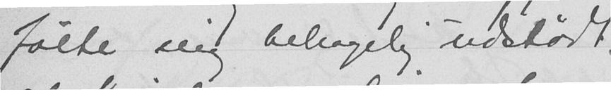følte mig behagelig udstødt
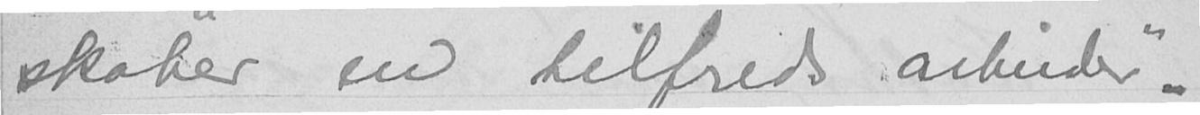skaber en tilfreds arbeider".
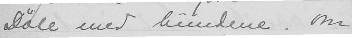Døle med hundene. Om
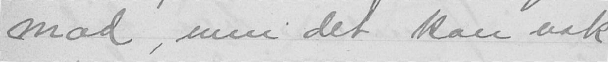mad, men det kan nok
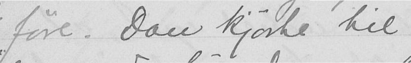føre. Don kjørte til
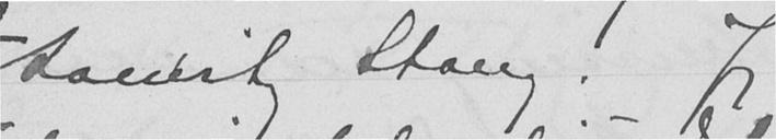Lauritz Stang. Jeg
